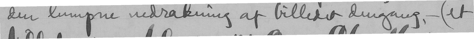den lumpne nedrakning af billedet dengang, - (et
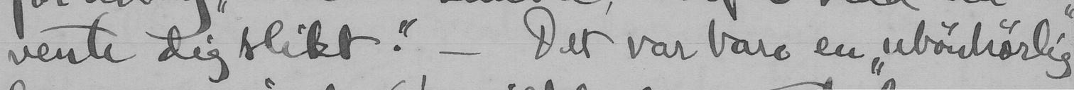vente dig slikt": - Det var bare en "ubønhørlig"
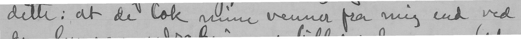dette: at de tok mine venner fra mig end ved
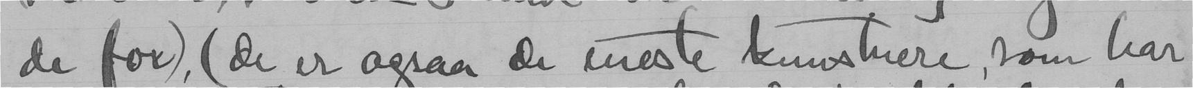de for), (de er ogsaa de eneste kunstnere, som har
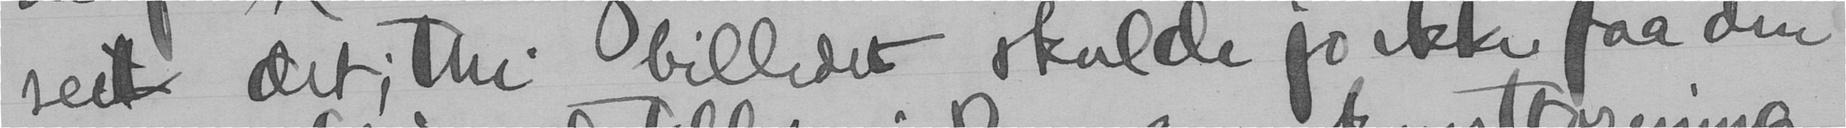seet det; thi billedet skulde jo ikke faa den
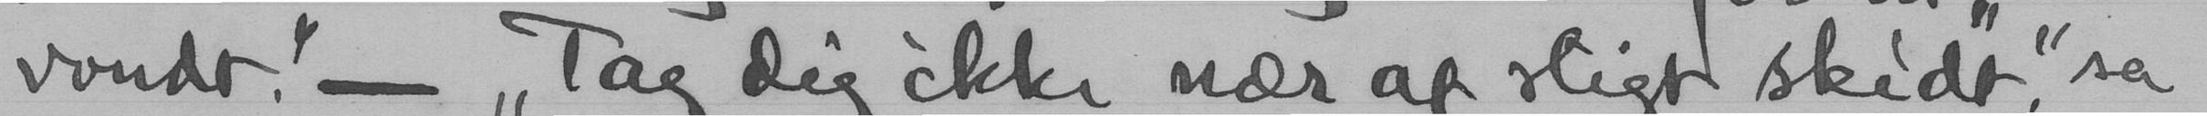vondt." - "Tag dig ikke nær af sligt skidt", sa
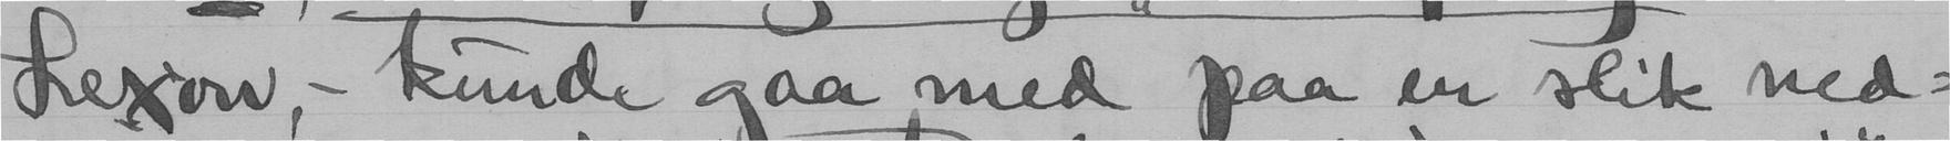Lexon, - kunde gaa med paa en slik ned-
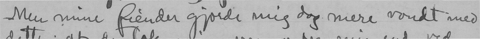Men mine fiender gjorde mig dog mere vondt med
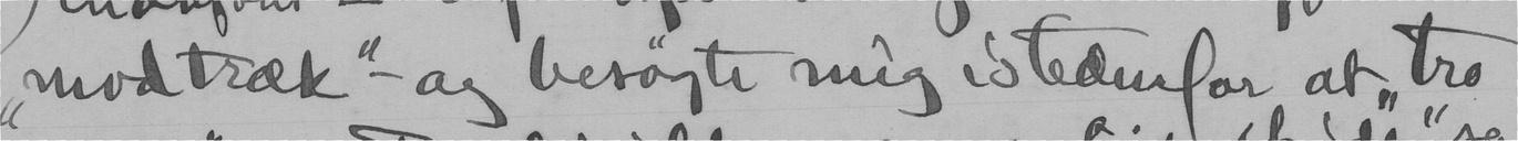"modtræk" - og besøgte mig istedenfor at "tro
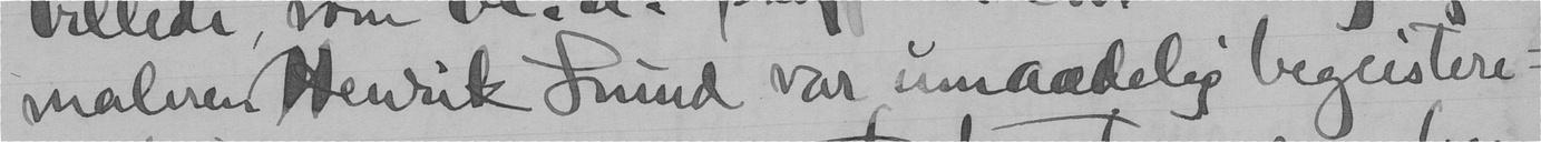maleren Henrik Lund var umaadelig begeistere-
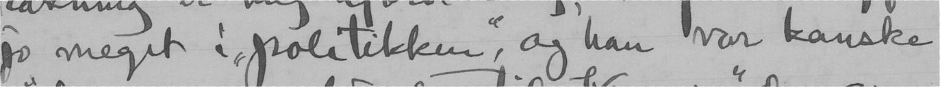jo meget i "politikken", og han var kanske
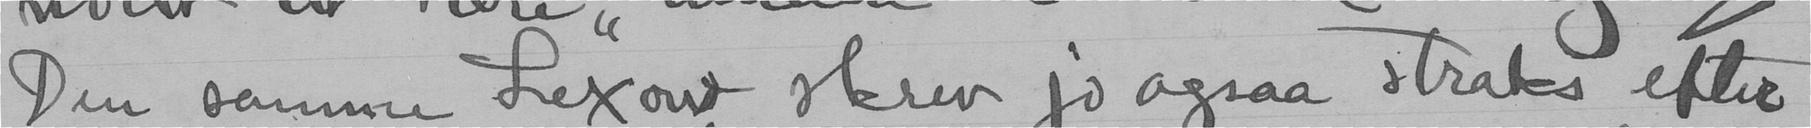Den samme Lexon skrev jo ogsaa straks efter
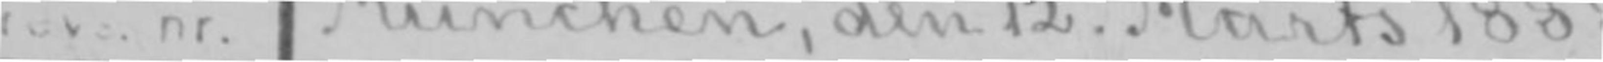München, den 12. Marts 1888.
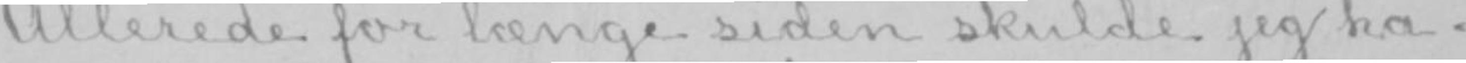Allerede for længe siden skulde jeg ha-
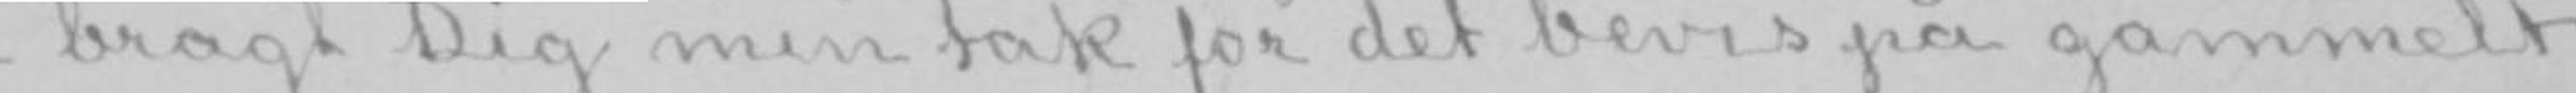ve bragt Dig min tak for det bevis på gammelt
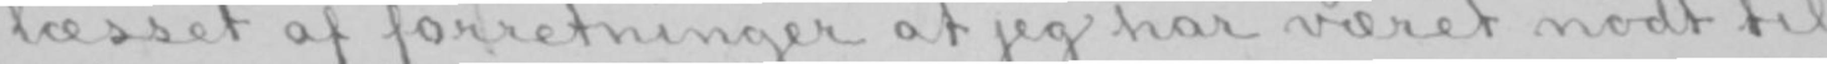læsset af forretninger at jeg har været nødt til
